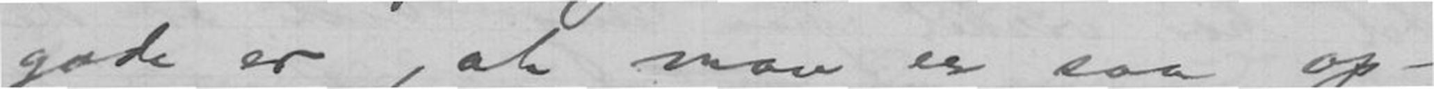gode er, at man er saa op-
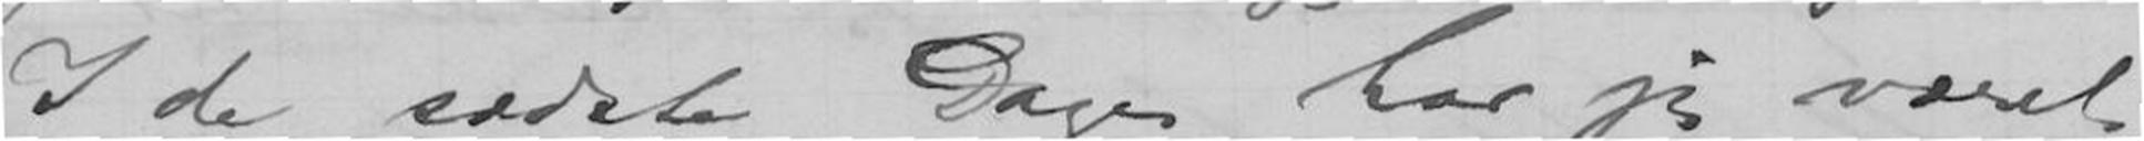I de sidste Dager har jeg været
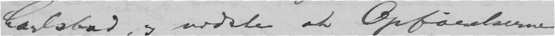Carlsbad, og vidste at Opførelserne
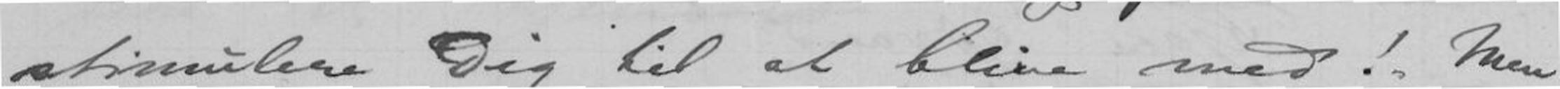stimulere Dig til at blive med! Men
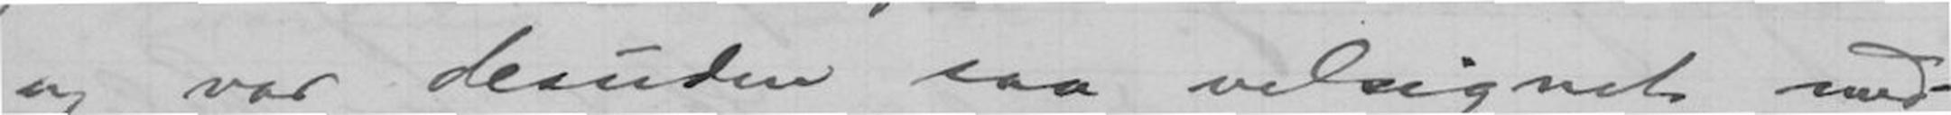og var desuden saa velsignet med-
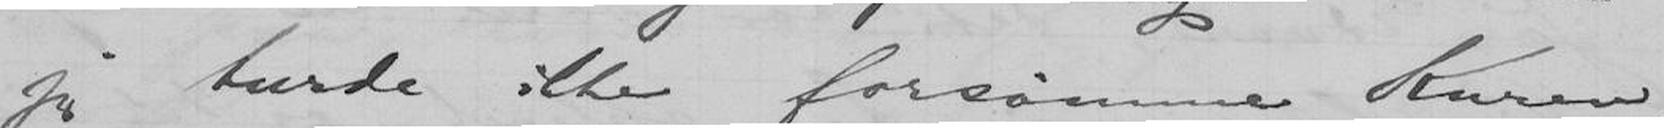jeg turde ikke forsømme Kuren,
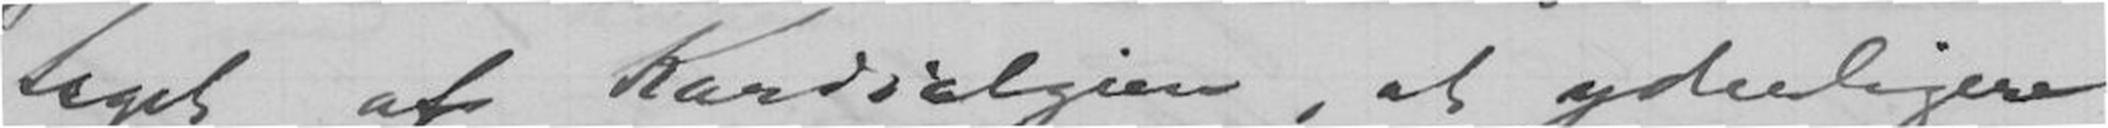taget af Kardialgien, at yderligere
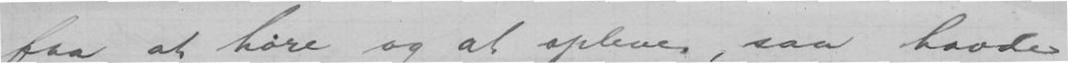faa at høre og at opleve, saa havde
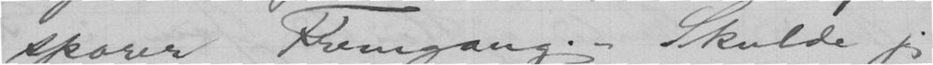sporer Fremgang. - Skulde jeg
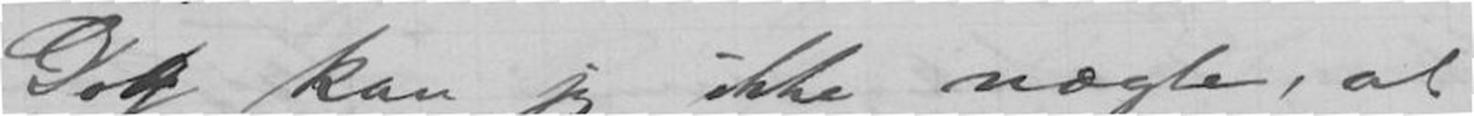Dog kan jeg ikke nægte, at
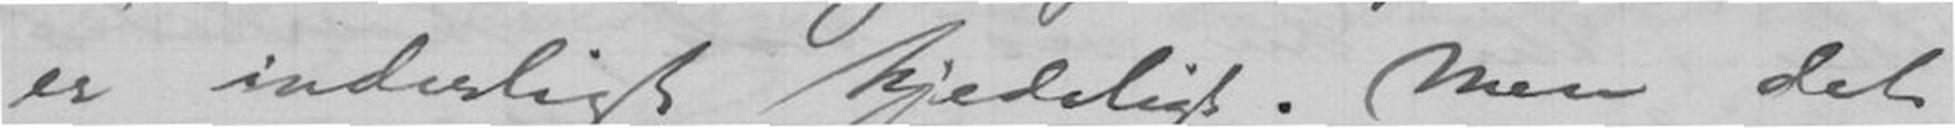er inderligt kjedeligt. Men det
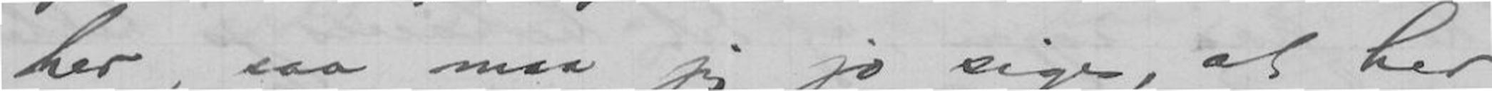her, saa maa jeg jo sige, at her
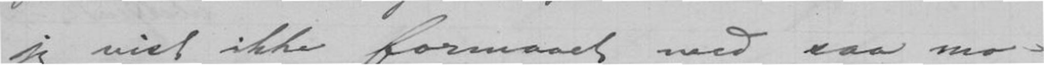jeg vist ikke formaaet med saa mo-
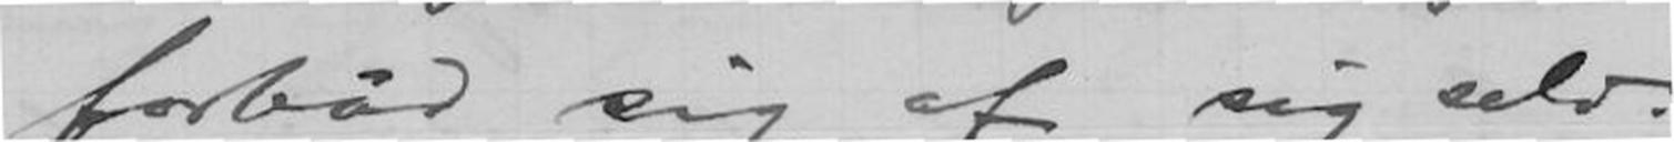forbød sig af sig selv.
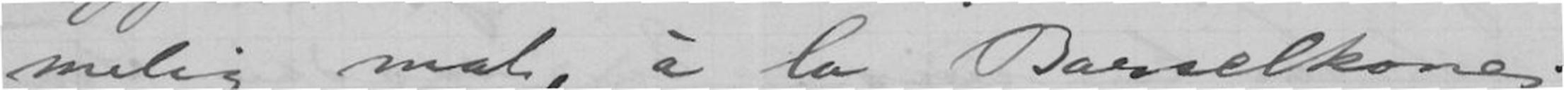melig mat, à la Barselkone.
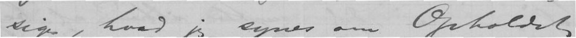sige, hvad jeg synes om Opholdet
